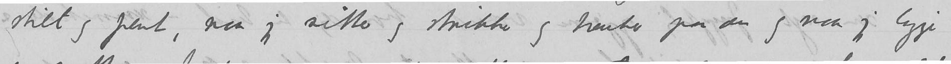stilt og pent, naar jeg sitter og strikker og tænker paa den og naar jeg ligger
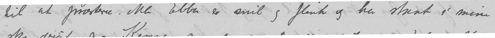til at præstere. Men Ebba er snil og flink og har staat i mine
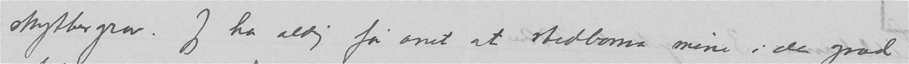skyttergrav.  Jeg har aldrig før anet at stedborna mine i den grad
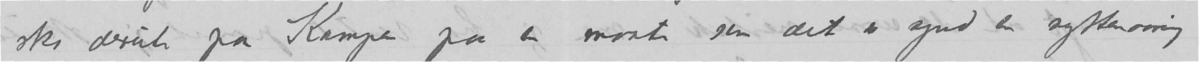sko derute paa Kampen paa en maate som det er synd en syttenåring
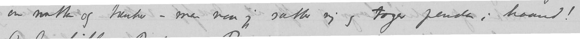om natten og tænker – men naar jeg satter mig og tager penden i haand!
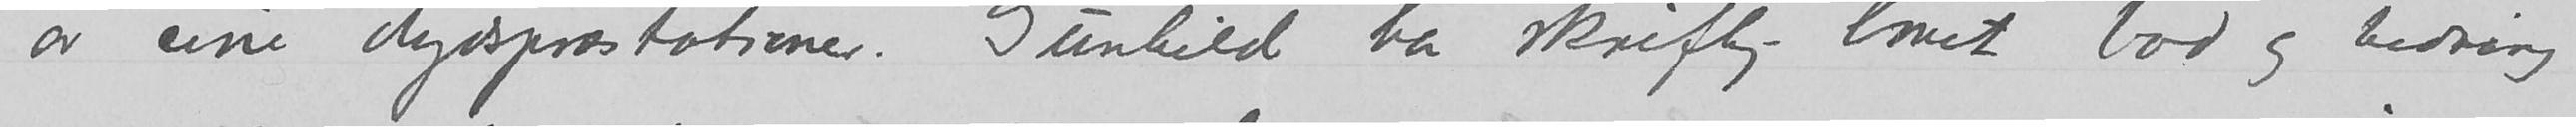av sine dydspræstationer. Gunhild har skriftlig lovet bod og bedring
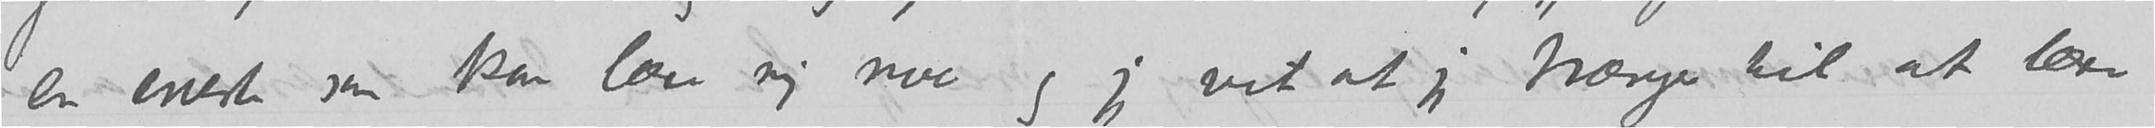en eneste som kan lære mig noe og jeg vet at jeg trænger til at lære
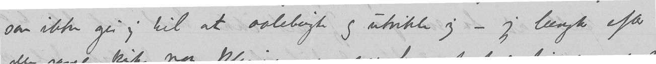sam ikke gir sig til at aalebugte og utvikle sig - jeg længter efter
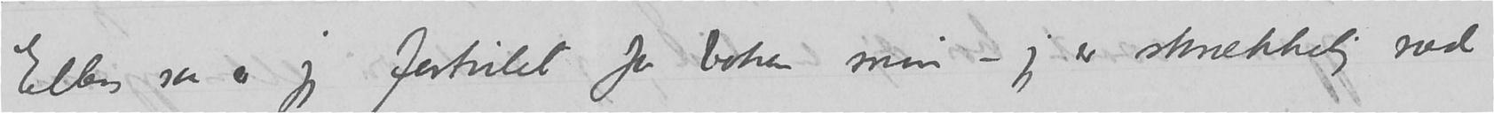Ellers saa er jeg fortvilet for boken min – jeg er skrækkelig ræd
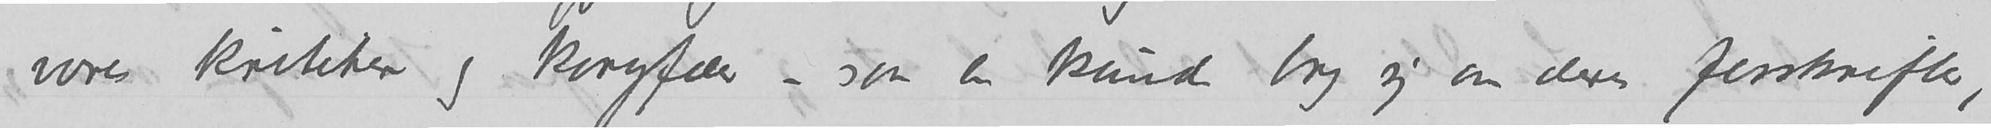vores kritiker og koryfæer – saa en kunde bry sig om deres forskrifter,
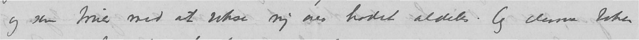og som truer med at vokse mig over hodet aldeles. Og denne boken
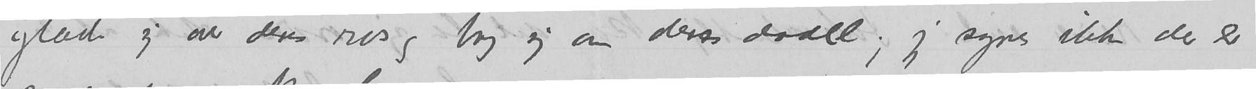glæde sig over deres ros og bry sig om deres dadel; jeg synes ikke der er
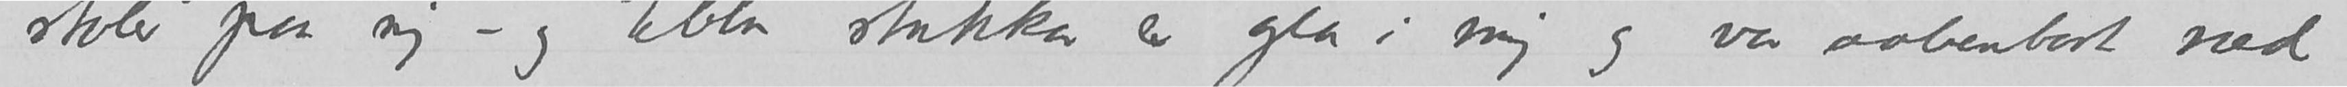stoler paa mig – og Ebba stakkar er gla i mig og var aabenbart ræd
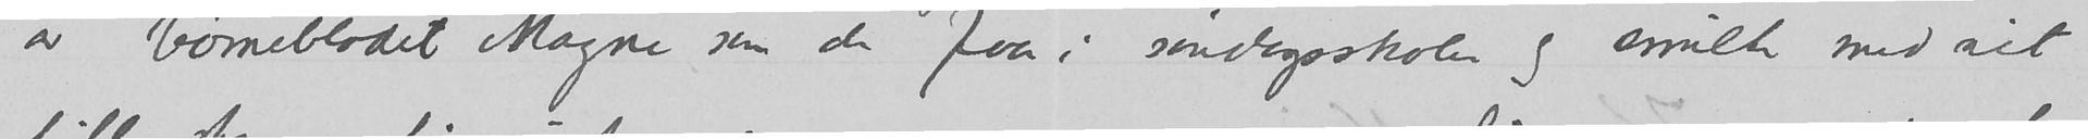av børneblodet Magne som de faar i søndagsskolen og smilte med sit
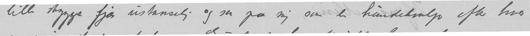lille stygge fjæs ustanselig og saa paa mig som en hundehvalp efter hver
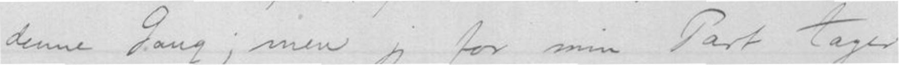denne Gang; men jeg for min Part tager
